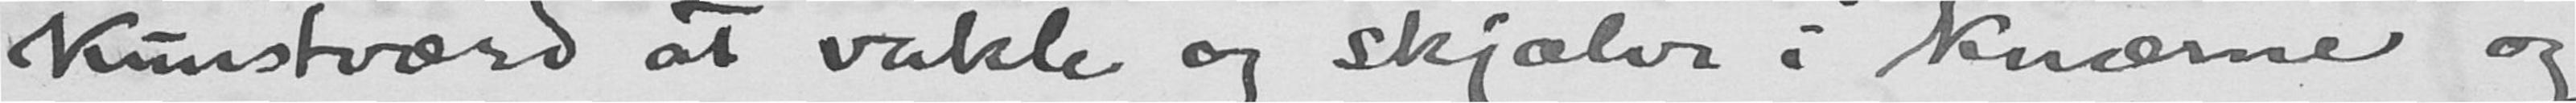kunstværd at vakle og skjælve i knærne og
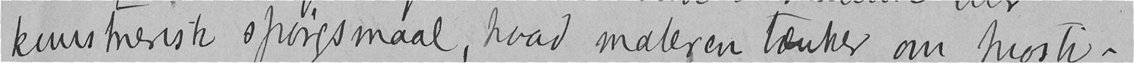kunstnerisk spørgsmaal, hvad maleren tænker om prosti-
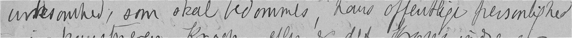virksomhed, som skal bedømmes, hans offentlige personlighed
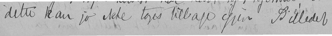dette kan jo ikke tages tilbage igjen. Billedet
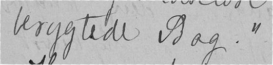berygtede Bog."
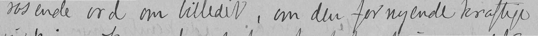rosende ord om billedet, om den fornyende kraftige
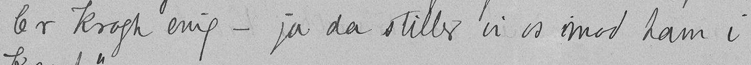Er Krogh enig - ja da stiller vi os imod ham i
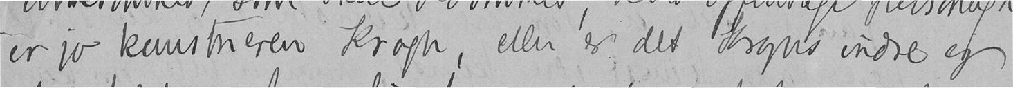er jo kunstneren Krogh, eller er det Kroghs indre og
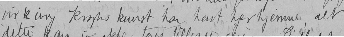virkning Kroghs kunst har havt herhjemme, alt
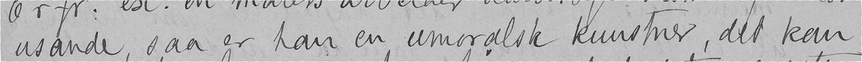usande, saa er han en umoralsk kunstner, det kan
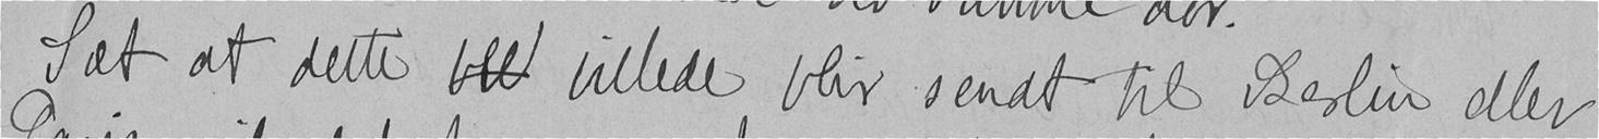Sæt at dette blev billede blir sendt til Berlin eller
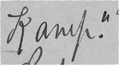Kamp."
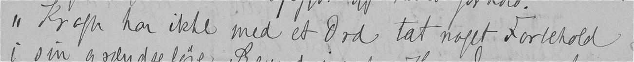"Krogh har ikke med et Ord tat noget Forbehold
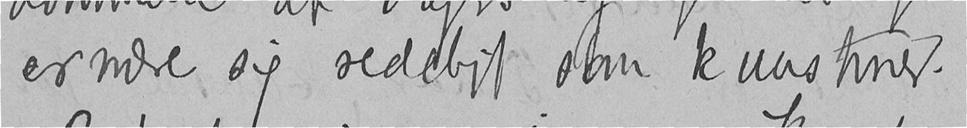ernære sig redeligt som kunstner.
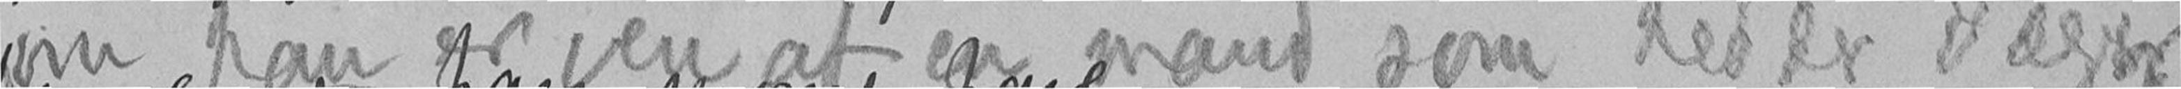om han er ven af en mand som heder Jæger.
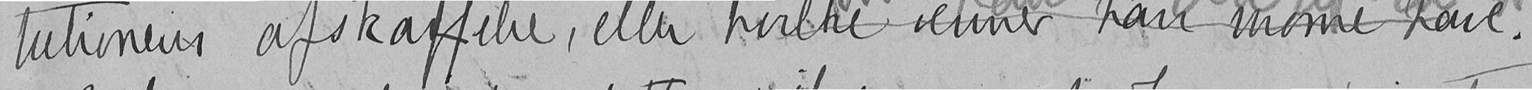tutionens afskaffelse, eller hvilke venner han monne have.
